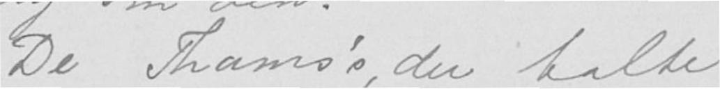De Thams's, du talte
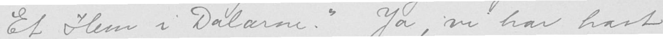"Et Hem i Dalarne". Ja, vi har havt
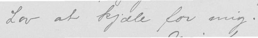Lov at kjæle for mig.
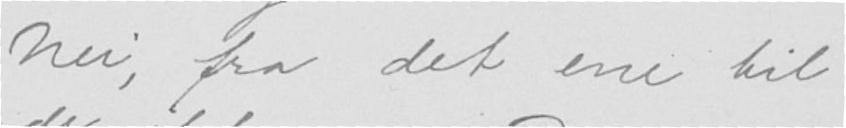Nei, fra det ene til
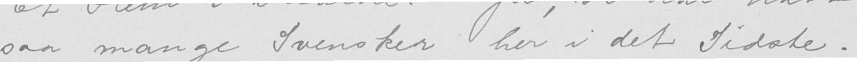saa mange svensker her i det Sidste.
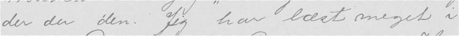der du den. Jeg har læst meget i
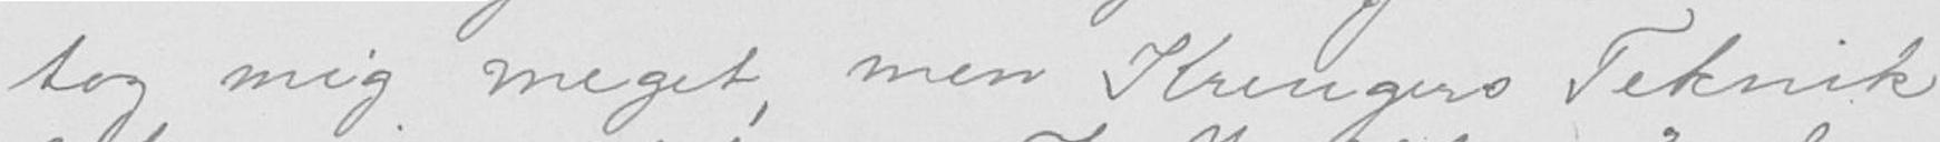tog mig meget, men Kreugers Teknik
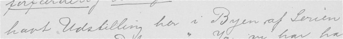havt Udstilling her i Byen af Serien
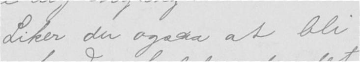Liker du ogsaa at bli
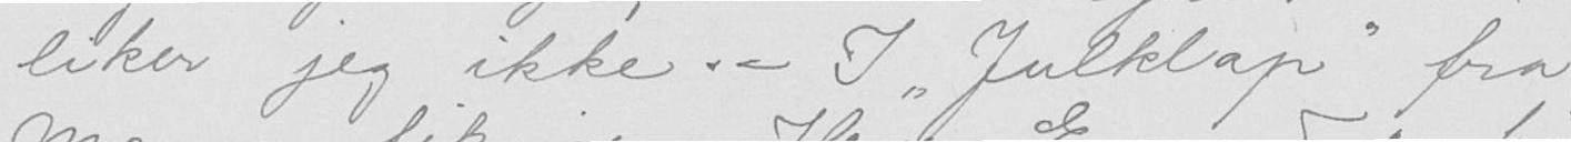liker jeg ikke. - I "Julklap" fra
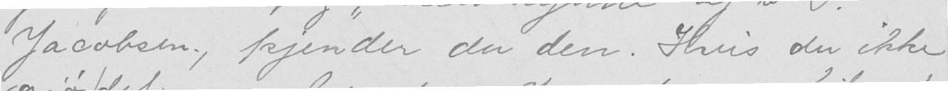Jacobsen, kjender du den. Hvis du ikke
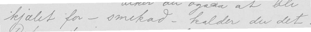kjælet for - smekad - kaller du det.
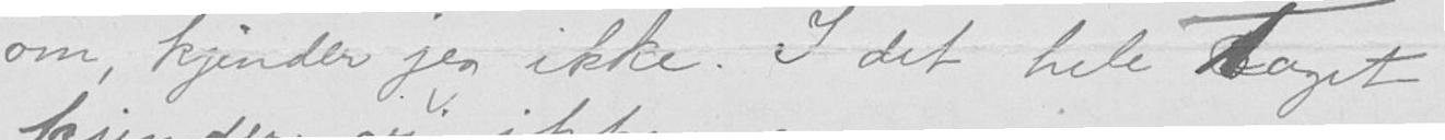om, kjender jeg ikke. I det hele taget
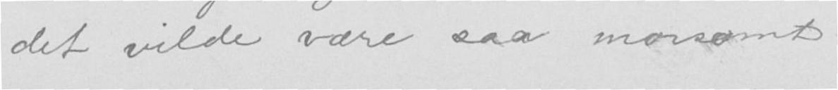det vilde være saa morsomt.
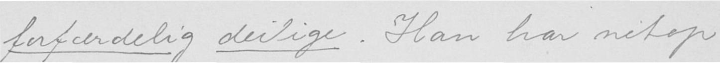forfærdelig deilige. Han har netop
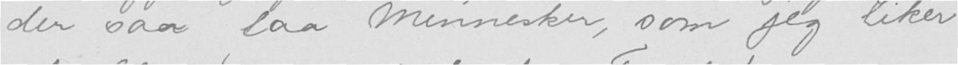der saa faa Mennesker, som jeg liker
